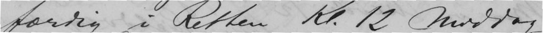færdig i Retten Kl. 12 Middag,
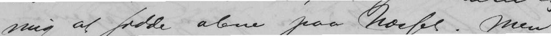mig at sidde alene paa Næsset. Men
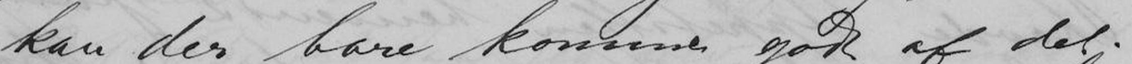kan der bare komme godt af det.
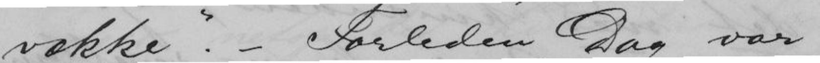vække". - Forleden Dag var
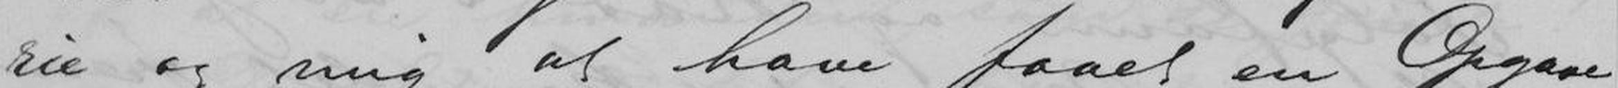rie og mig at have faaet en Opgave,
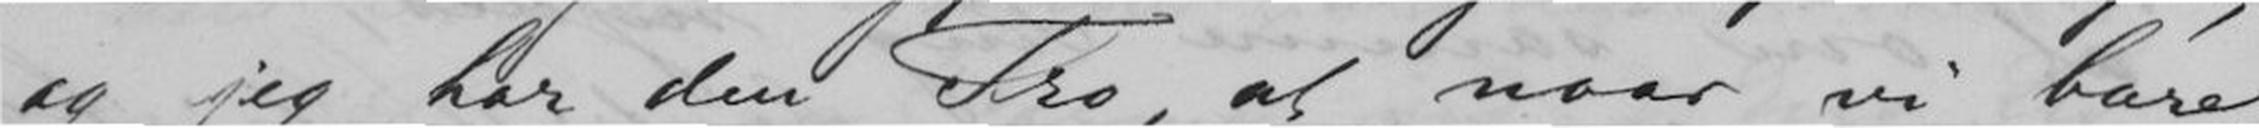og jeg har den Tro, at naar vi bare
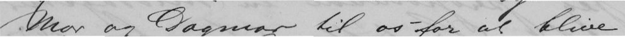Mor og Dagmar til os for at blive
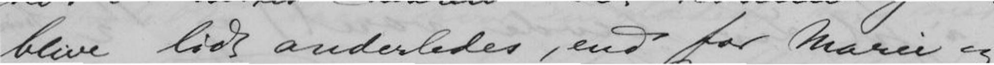blive lidt anderledes, end for Marie og
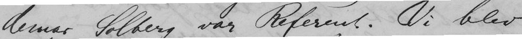demar Solberg var Referent. Vi blev
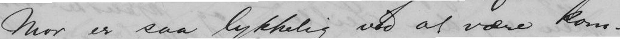Mor er saa lykkelig ved at være kom-
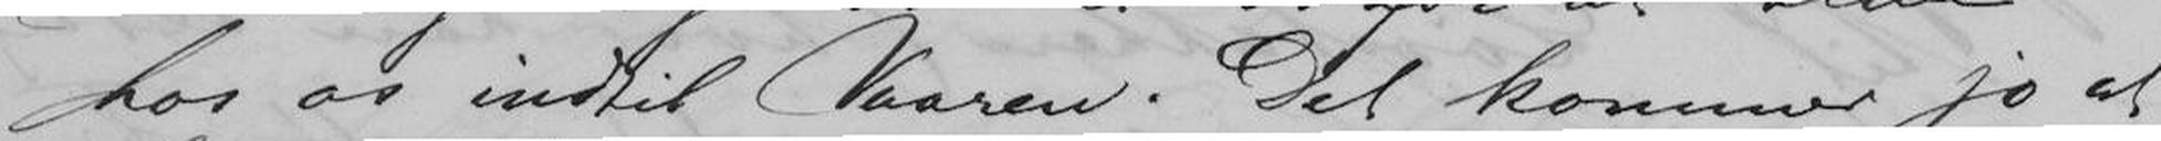hos os indtil Vaaren. Det kommer jo at
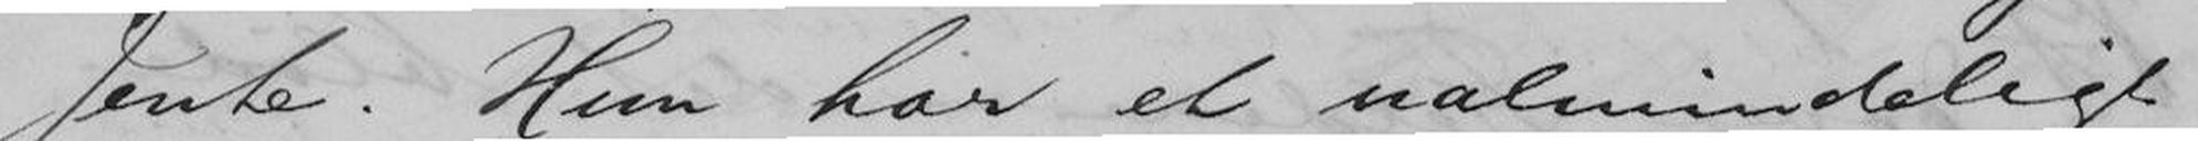Jente. Hun har et ualmindeligt
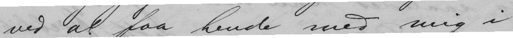ved at faa hende med mig i
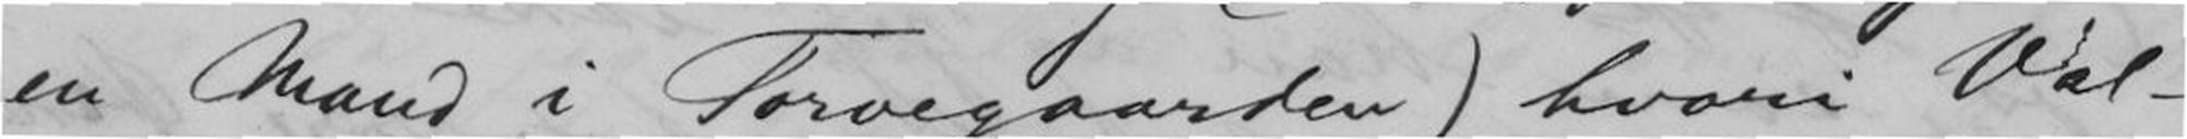en Mand i Torvegaarden) hvori Val-
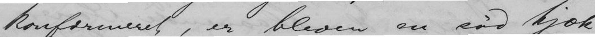konfirmeret, er bleven en sød kjæk
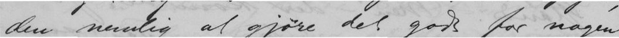den nemlig at gjøre det godt for nogen,
